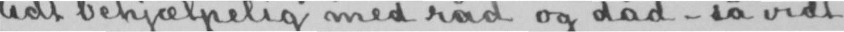lidt behjælpelig med råd og dåd- så vidt
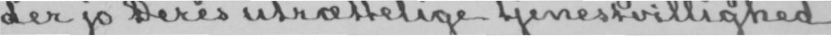der jo Deres utrættelige tjenestvillighed
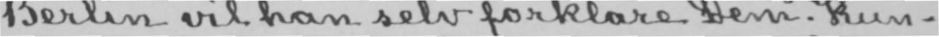Berlin vil han selv forklare Dem. Kun-
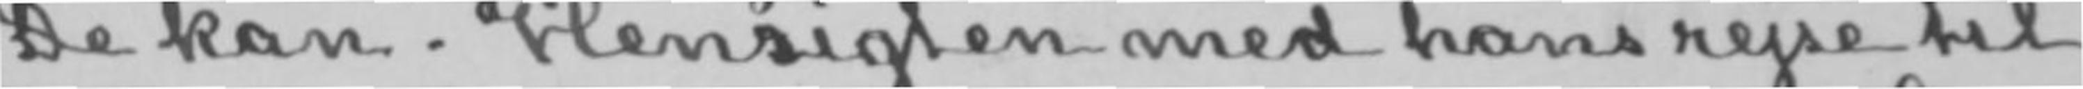De kan. Hensigten med hans rejse til
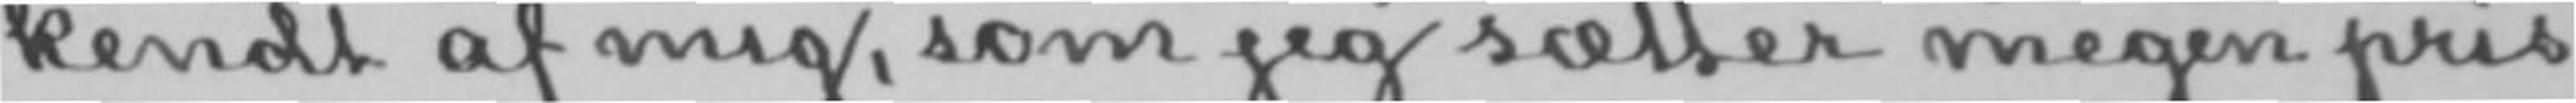kendt af mig, som jeg sætter megen pris
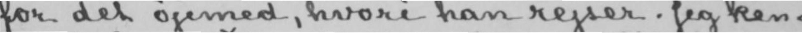for det øjemed, hvori han rejser. Jeg ken-
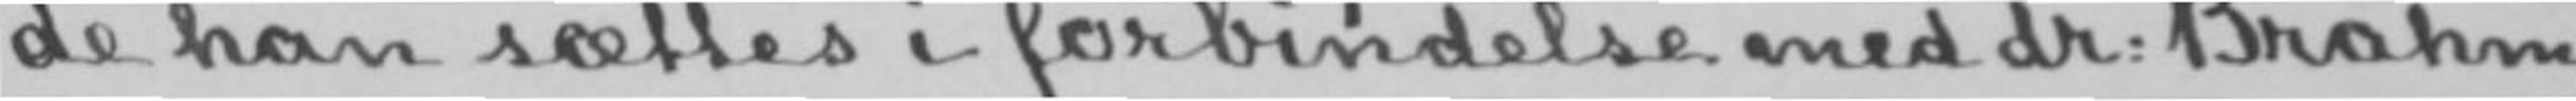de han sættes i forbindelse med dr: Brahm,
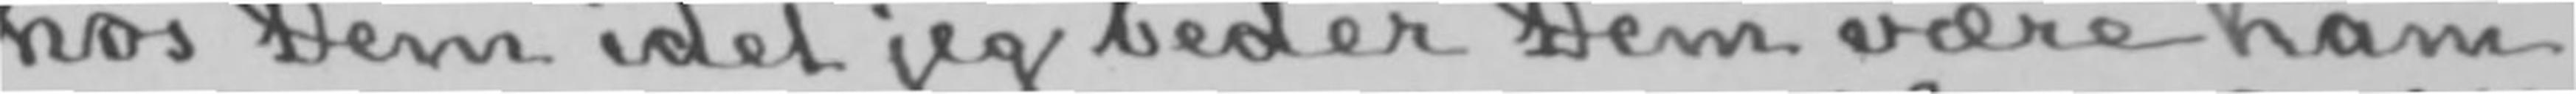hos Dem idet jeg beder Dem være ham
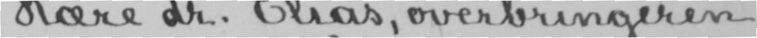Kære dr. Elias, overbringeren
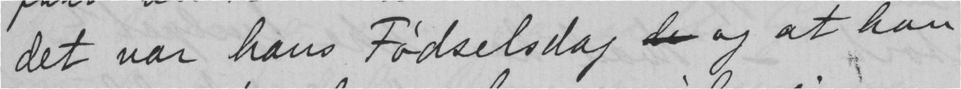det var hans Fødselsdag  og at han
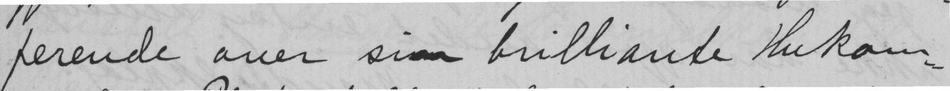ferende over sin brilliante Hukom-
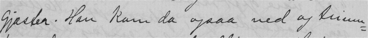Gjæster. Han kom da ogsaa ned og trium-
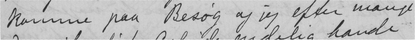komme paa Besøg og jeg efter mange
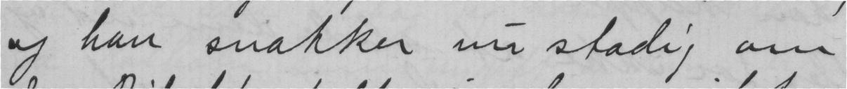og han snakker nu stadig om
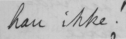han ikke!
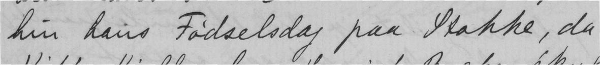hin hans Fødselsdag paa Stokke, da
